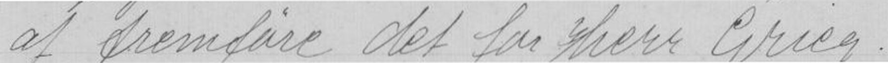at fremföre det for Herr Grieg.
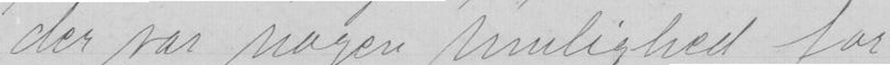der var nogen mulighed for
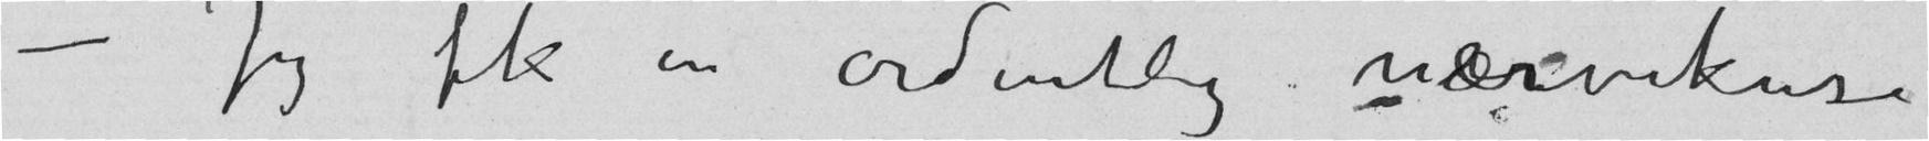– Jeg fik en ordentlig nærvekur
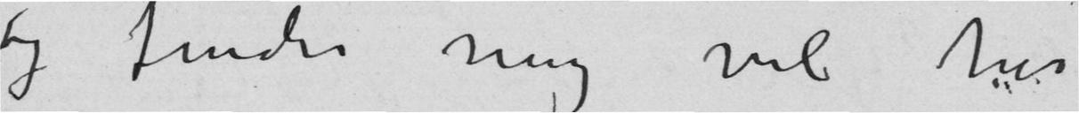og finder mig vel her i
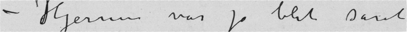– Hjernen var jo blit såret
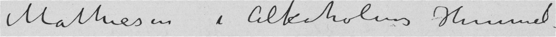Mathiesen i Alkoholens Himmel –
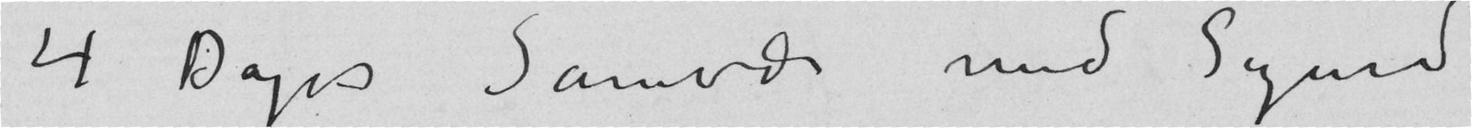4 Dages Samvær med Sigurd
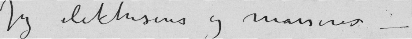Jeg elektriseres og masseres –
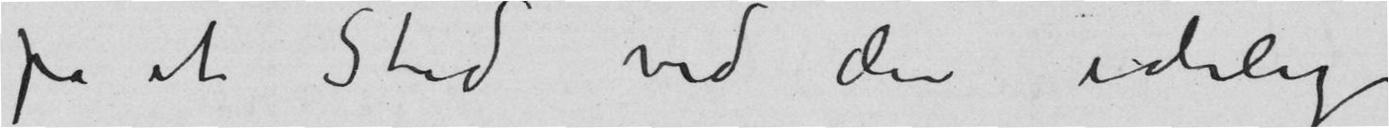på et sted ved den idelige
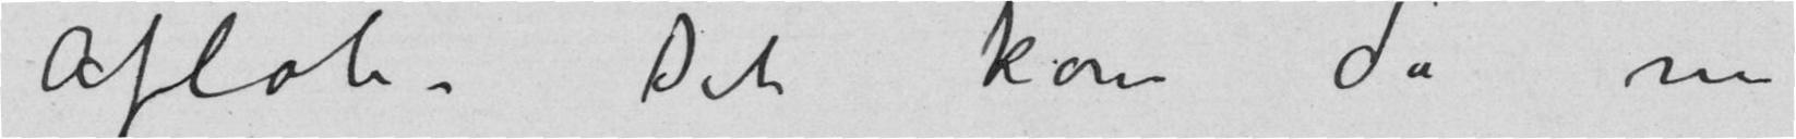Afløb. Det kom da nu
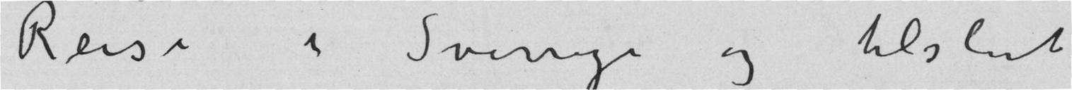Reise i Sverige og tilslut
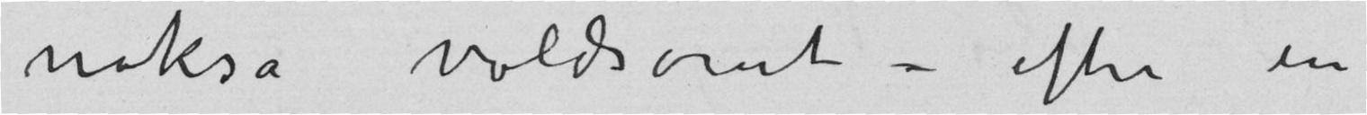nokså voldsomt – efter en
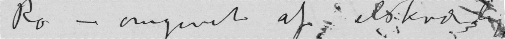Ro – omgivet af elskværdige
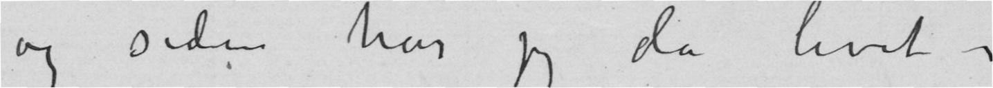og siden har jeg da levet i
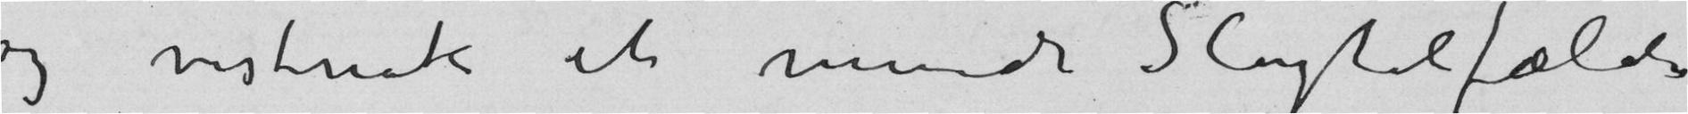og vistnok et mindre Slagtilfælde
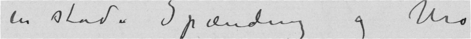en stadig Spænding og Uro
